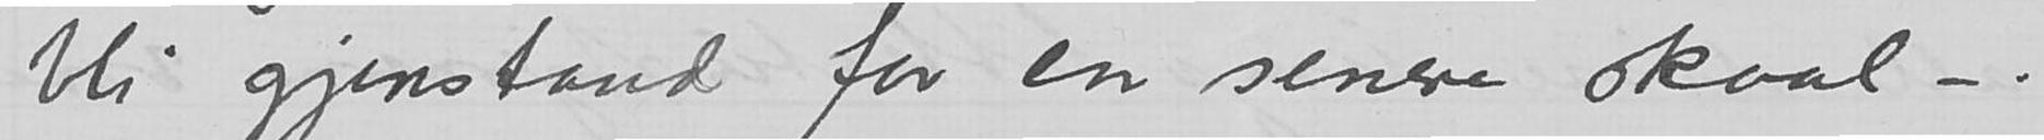bli gjenstand for en senere skaal -
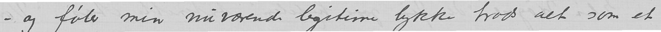og føler min nuværende legitime lykke trods alt som et
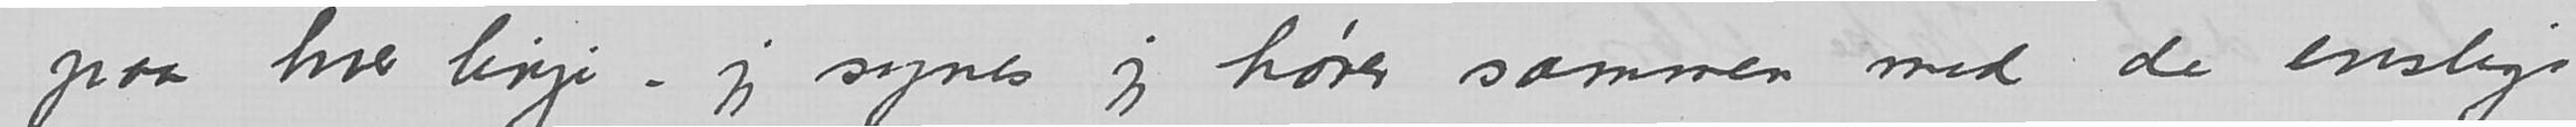paa hver linje - jeg synes jeg hører sammen med de enslige
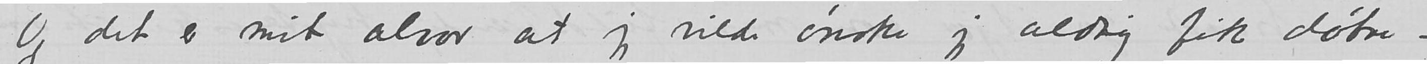Og det er mit alvor at jeg vilde ønske jeg aldrig fik døtre -.
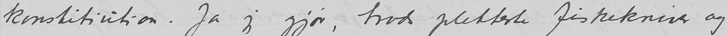konstitusjon. Ja jeg gjør, trods plettete fiskekniver og
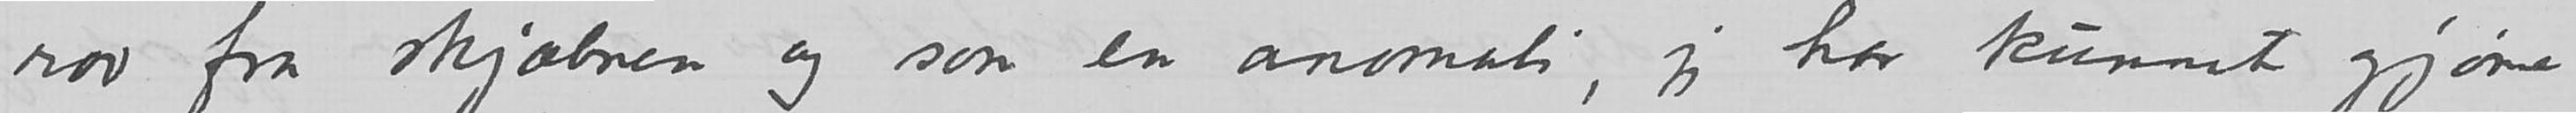rov fra skjæbnen og som en anomali, jeg har kunnet gjøre
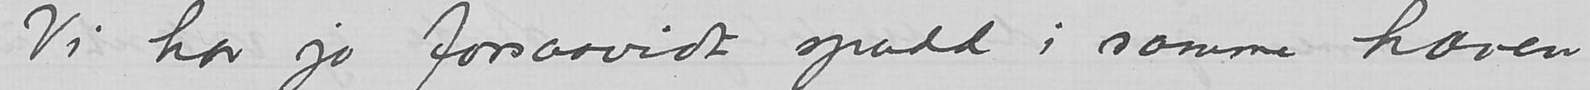Vi har jo forsaavidt spadd i samme haven
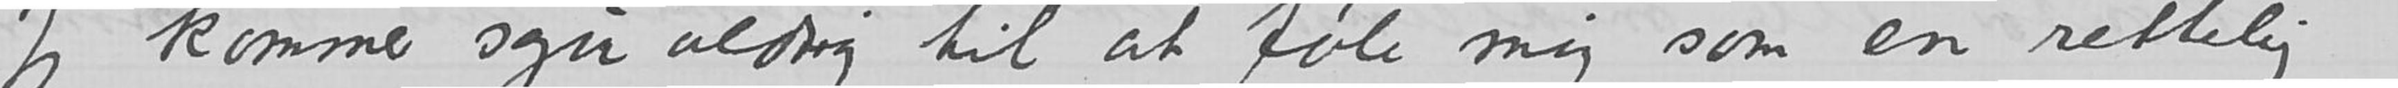Jeg kommer sgu aldrig til at føle mig som en rettelig
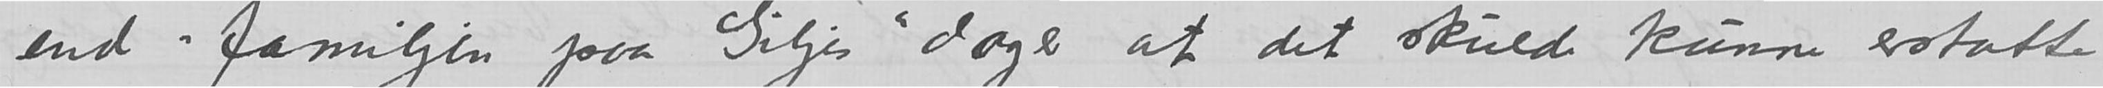end «familjen paa Giljes» dager at det skulde kunne erstatte
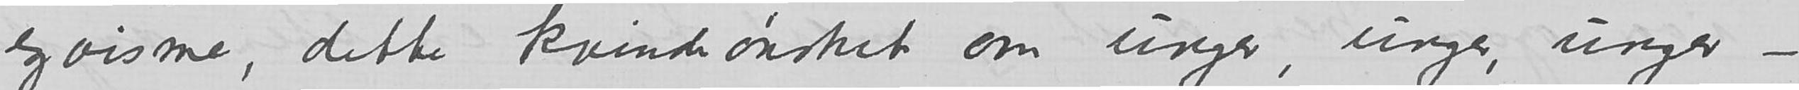av egoisme, dette kvindeønsket om unger, unger, unger -
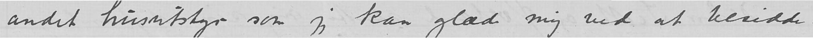andet husutstyr som jeg kan glæde mig ved at besidde.
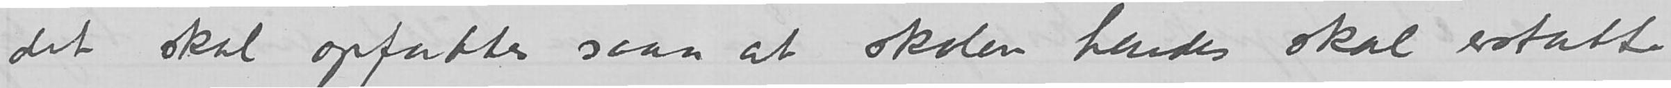det skal opfattes saan at skolen hendes skal erstatte
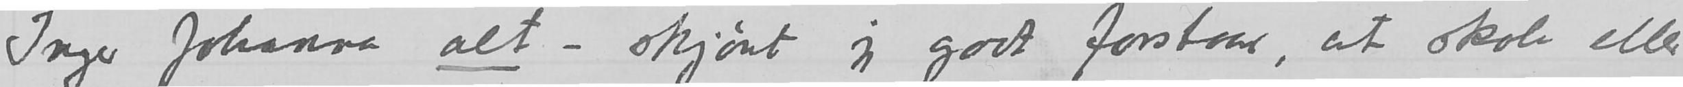Inger Johanne alt - skjønt jeg godt forstaar, at skole eller
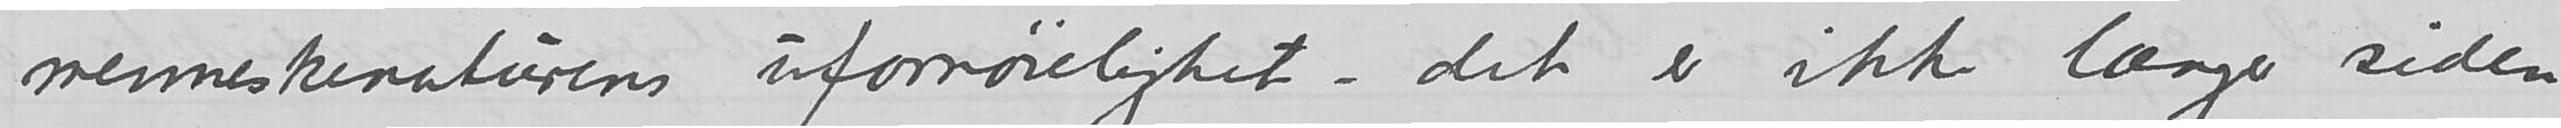menneskenaturens ufornøielighet - det er ikke længer siden
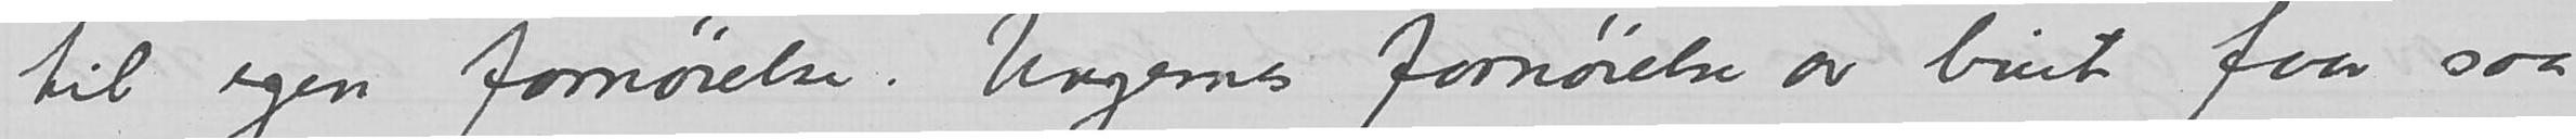til egen fornøielse. Ungernes fornøielse av livet faar saa
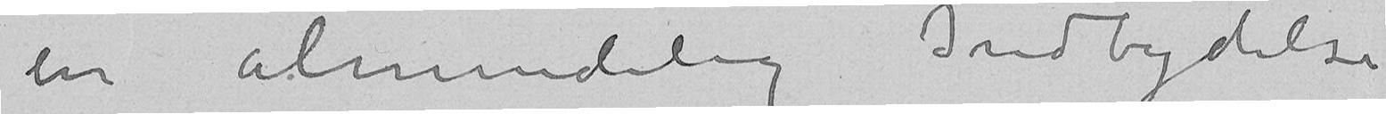en almindelig Indbydelse
